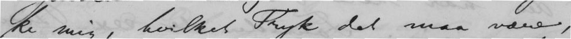ke mig, hvilket Tryk det maa være,
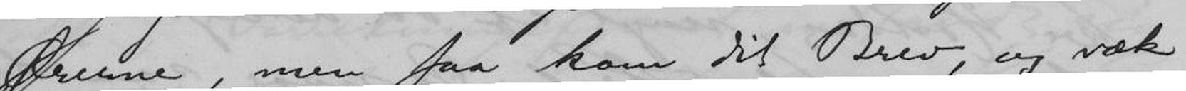Ørene, men saa kom dit Brev, og væk
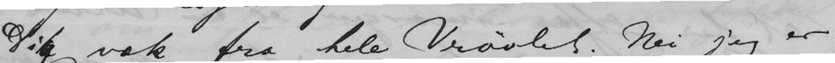Dig væk fra hele Vrøvlet. Nei jeg er
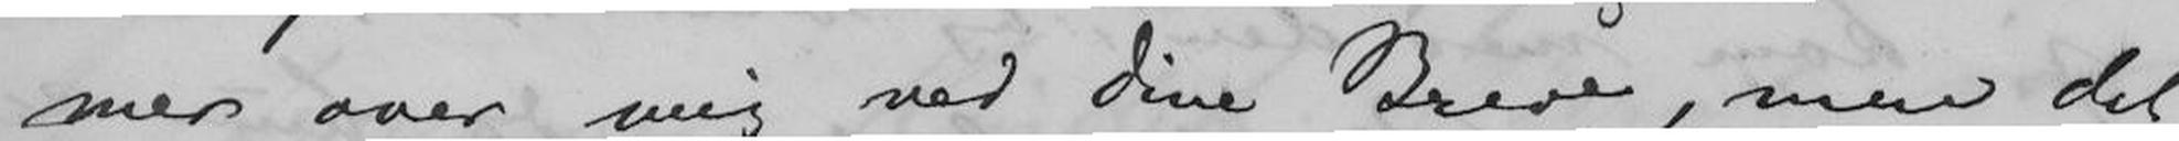mer over mig ved dine Breve, men det
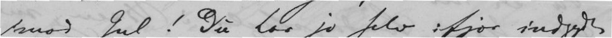mod Jul! Du har jo selv ifjor indgydt
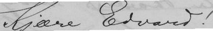Kjære Edvard!
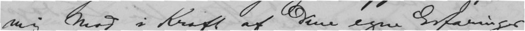mig Mod i Kraft af Dine egne Erfaringer
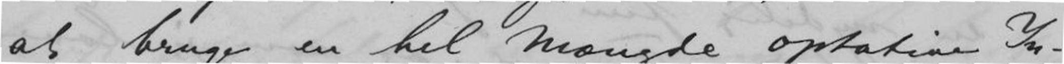at bringe en hel Mængde optative In-
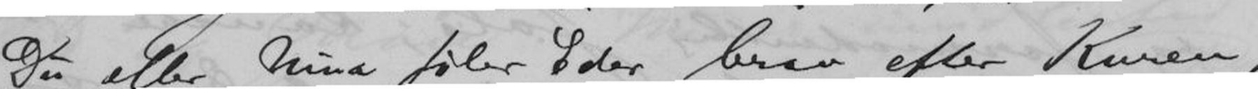Du eller Nina føler Eder bra efter Kuren,
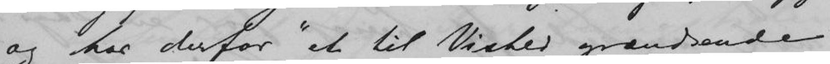og har derfor "et til Vished grændsende
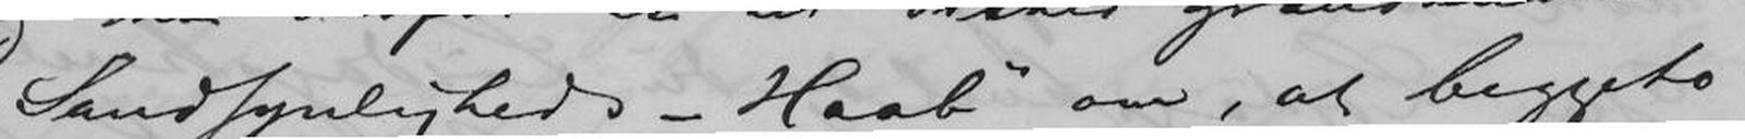Sandsynligheds-Haab" om, at beggeto
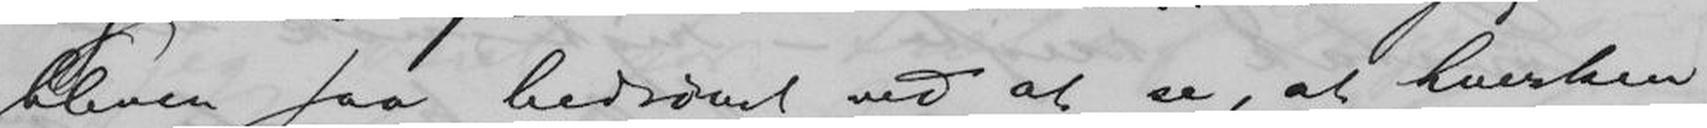bleven saa bedrøvet ved at se, at hverken
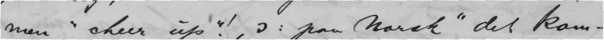men "cheer up"!, ): paa Norsk "det kom-
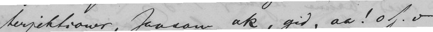terjektioner, saasom ak, gid, aa! o.s.v
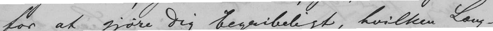for at gjøre Dig begribeligt, hvilken Læng-
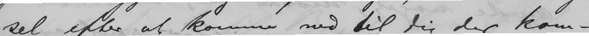sel efter at komme ned til Dig der kom-
